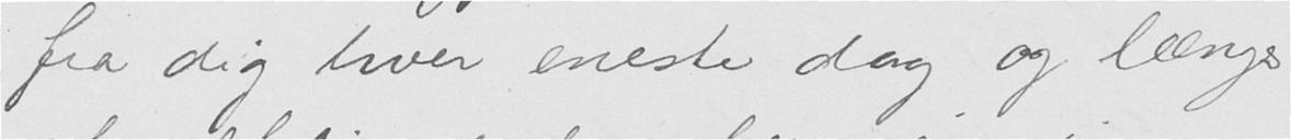fra dig hver eneste dag og længes
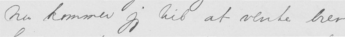Nu kommer jeg til at vente brev
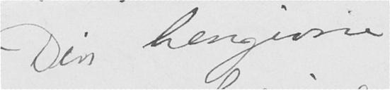Din hengivne
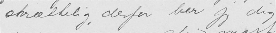skrækkelig, derfor ber jeg dig
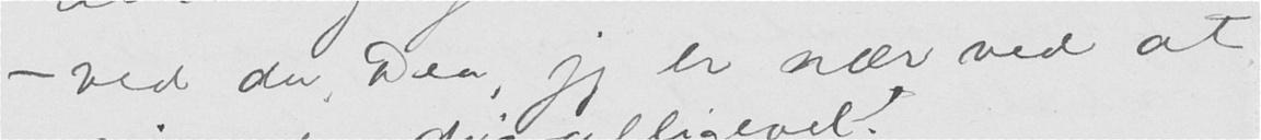- ved du, Dea, jeg er nær ved at
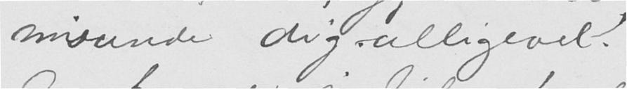misunde dig alligevel!
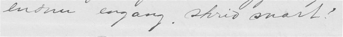endnu engang, skriv snart!
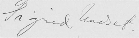Sigrid Undset.
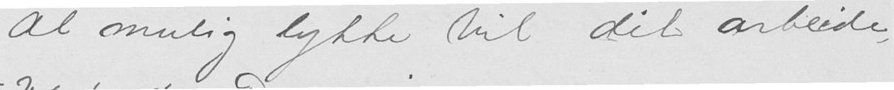Al mulig lykke til dit arbeide,
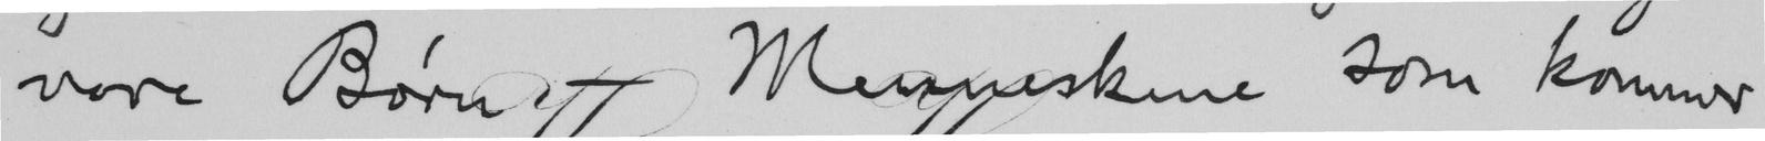vore Børn - Menneskene som kommer
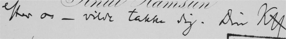efter os - vilde takke dig. Din KH
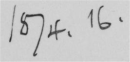18/4. 16.
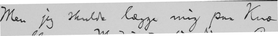Men jeg skulde lægge mig paa Knæ
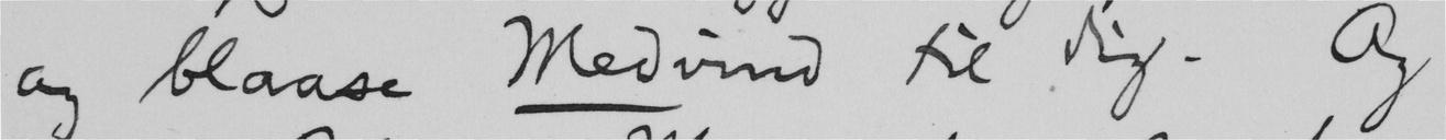og blaase Medvind til dig. Og
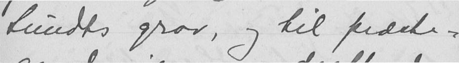Sundts grav, og til præste-
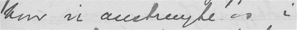hvor vi anstrengte os i
